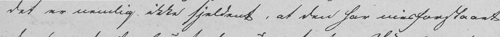det er nemlig ikke sjeldent, at den har misforstaaet
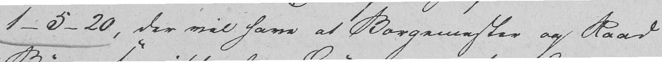1-3-26, der vil have at Borgemester og Raad
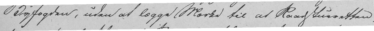Byfogden, uden at lægge Mærke til at Raadstueretten,
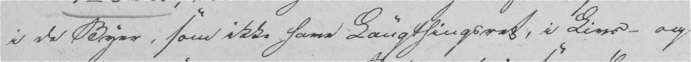i de Byer, som ikke have Laugthingsret, i Livs- og
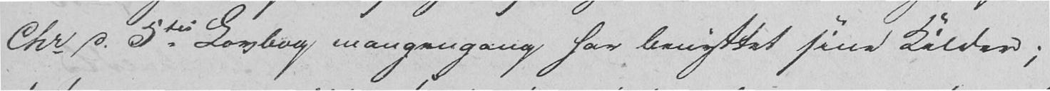Chr d. 5tes Lovbog mangengang har benyttet sine Kilder;
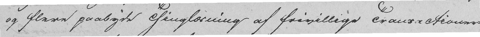og flere paabyde Thinglæsning af frivillige Transactioner
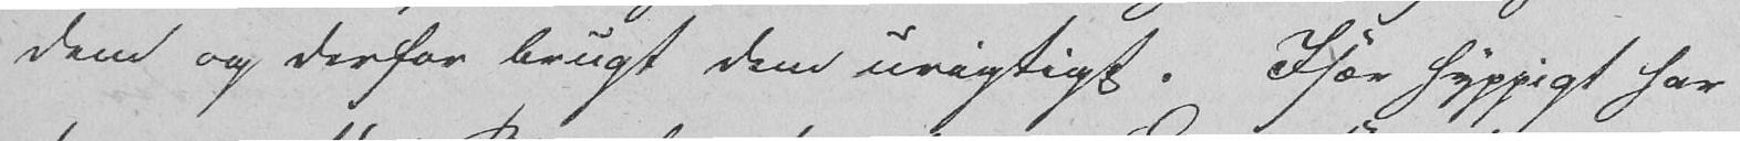dem og derfor brugt dem urigtigt. Især hyppigt har
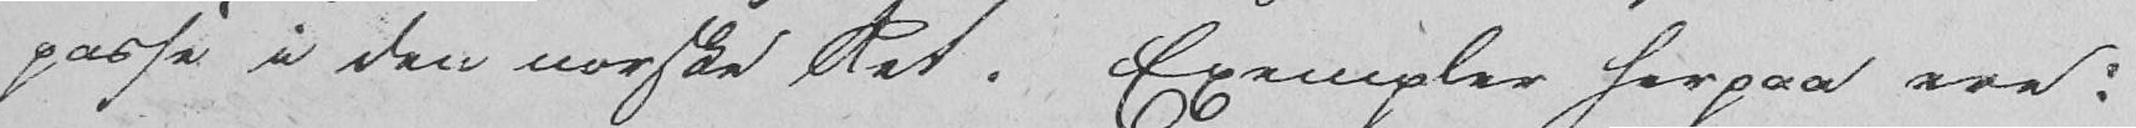passe i den norske Ret. Exempler herpaa ere:
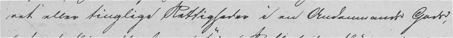ret eller tinglige Rettigheder i en Andenmands Gods,
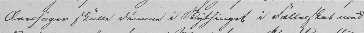Æressager skulle dømme i Bythinget i Fællesskab med
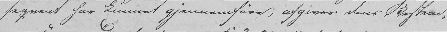seqvent har kunnet gjennemføre, afgiver dens Bestem-
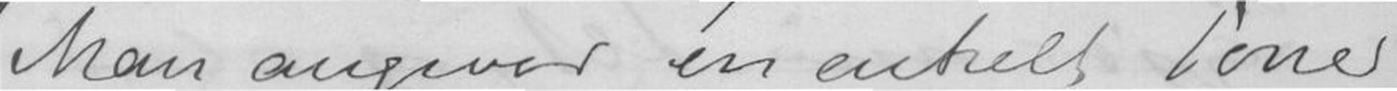Man angiver en enkelt Tone
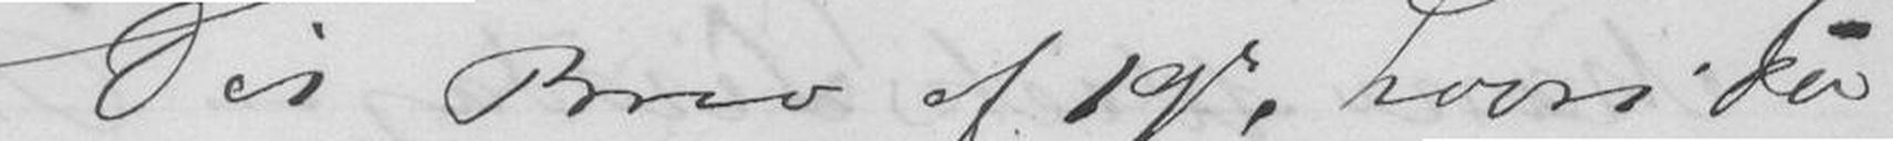Dit Brev af 19de, hvori Du
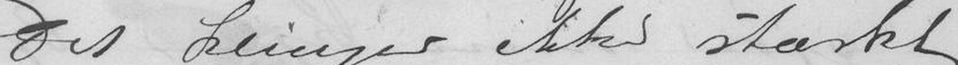Det klinger ikke stærkt
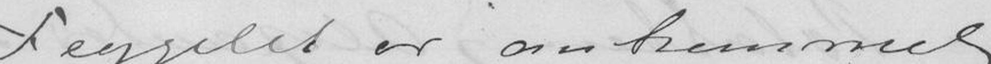Flygelet er ankommet
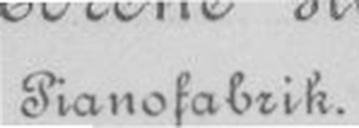Pianofabrik.
